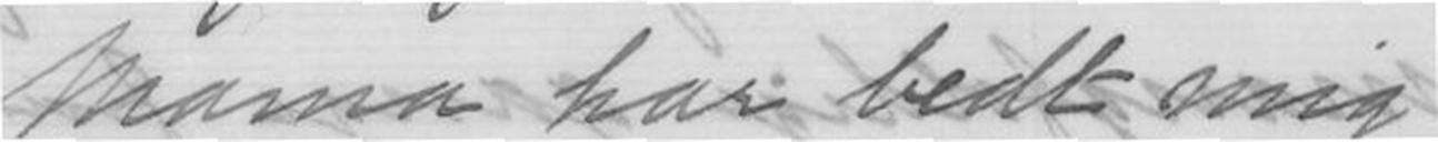Mamma har bedt mig
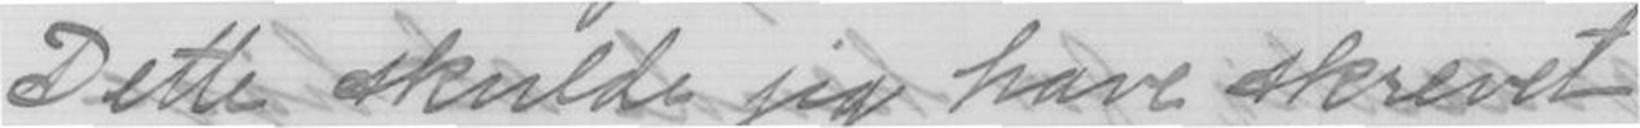Dette skulde jeg have skrevet
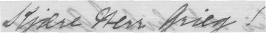Kjære Herr Grieg!
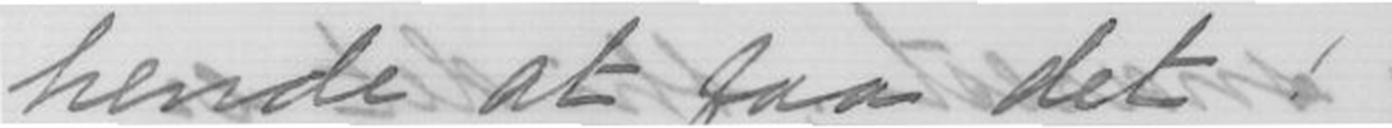hende at faa det!
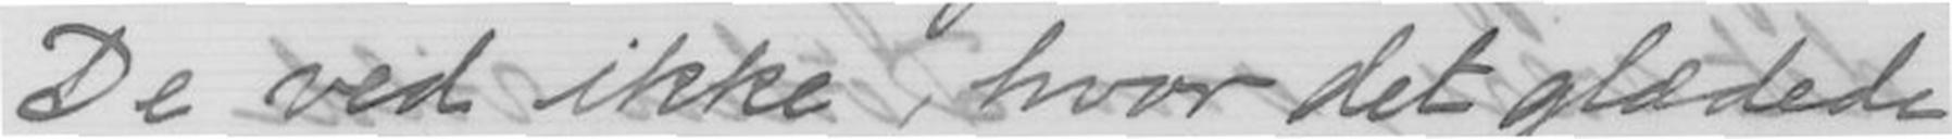De ved ikke, hvor det glædede
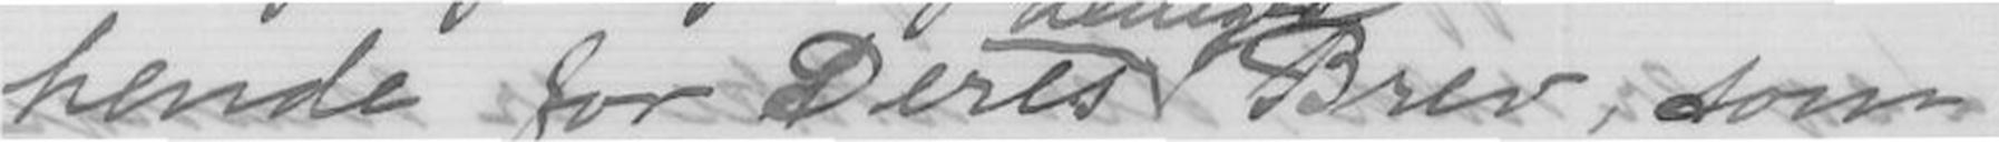hende for Deres Brev, dom
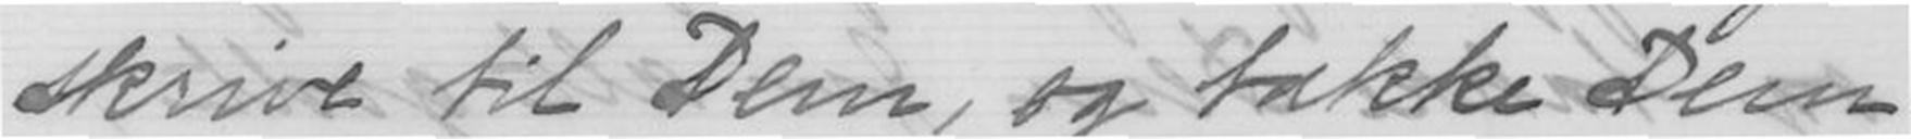skrive til Dem, og takke Dem
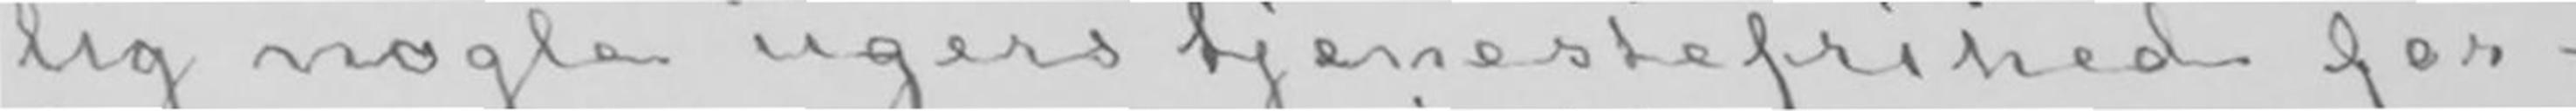lig nogle ugers tjenestefrihed for-
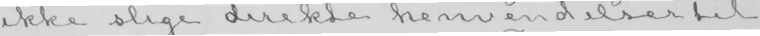ikke slige direkte henvendelser til
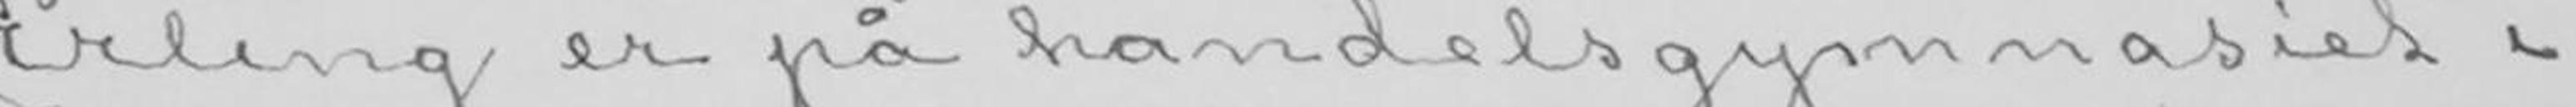Erling er på handelsgymnasiet i
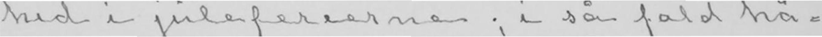hid i juleferierne; i så fald hå-
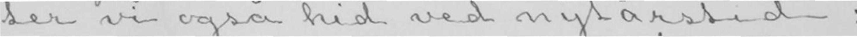ter vi også hid ved nytårstid;
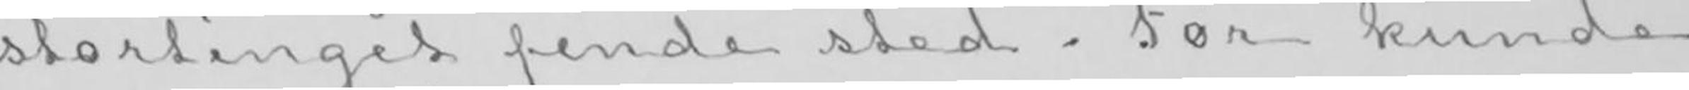stortinget finde sted. Før kunde
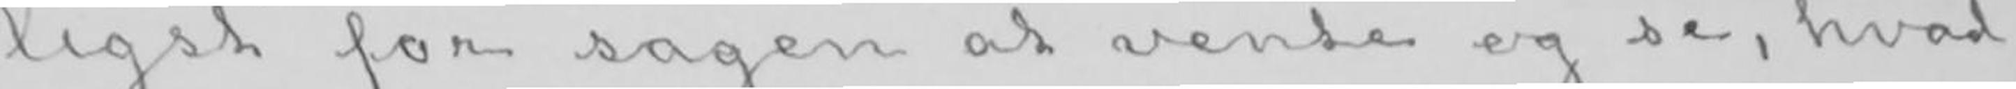ligst for sagen at vente og se, hvad
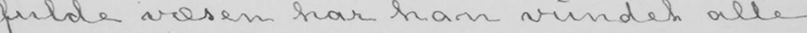fulde væsen har han vundet alle
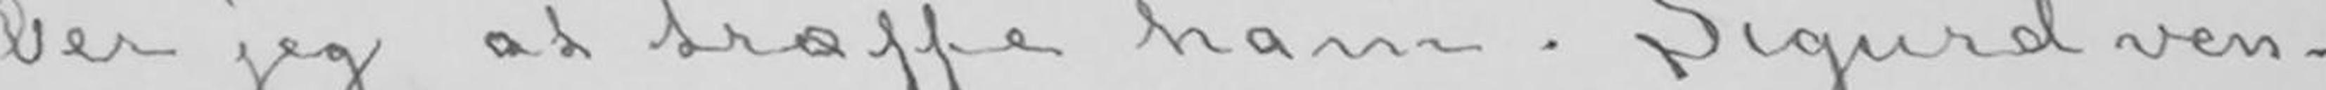ber jeg at træffe ham. Sigurd ven-
